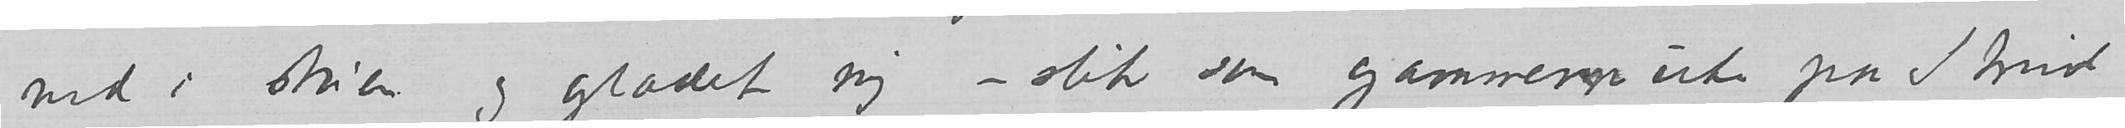ned i stuen og glædet mig – slik som gammern ute paa Strid
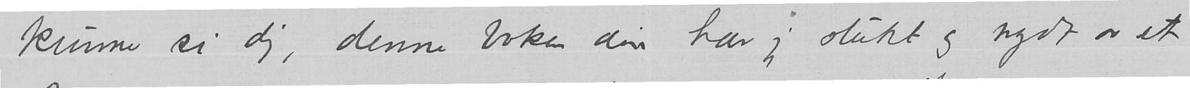kunne si dig, denne boken din har jeg slukt og nydt av et
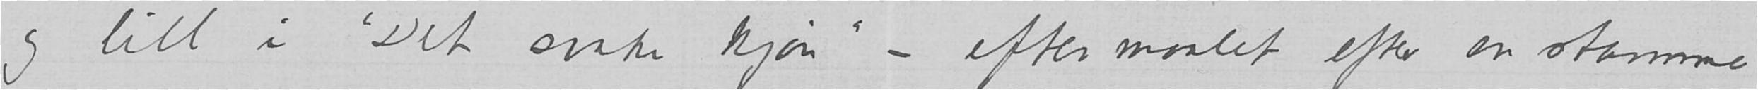og litt i «Det svake kjøn» – eftermaalet efter en stamme
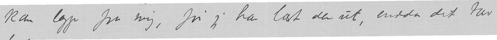kan legge fra mig, før jeg har læst den ut, endda det tar
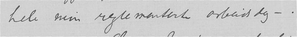hele min reglementerte arbeidsdag –.
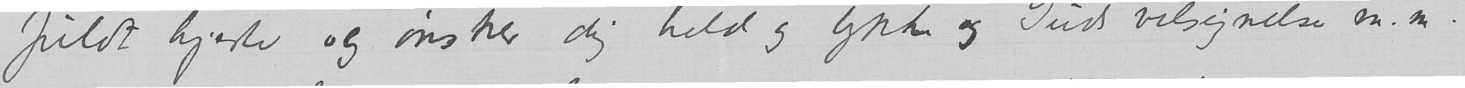fuldt hjerte og ønsker dig held og lykke og Guds velsignelse m. m.
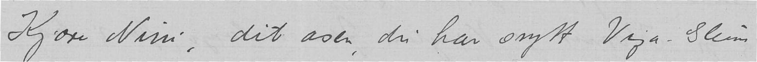Kjære Nini, dit asen, du har snytt Viga-Glum
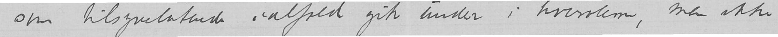som tilsynelatende ialfald gik under i hvirvlerne, men ikke
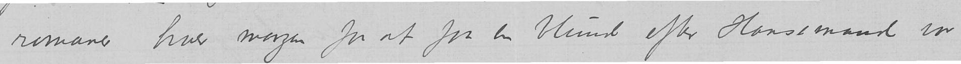romaner hver morgen for at faa en blund efter Hansemand var
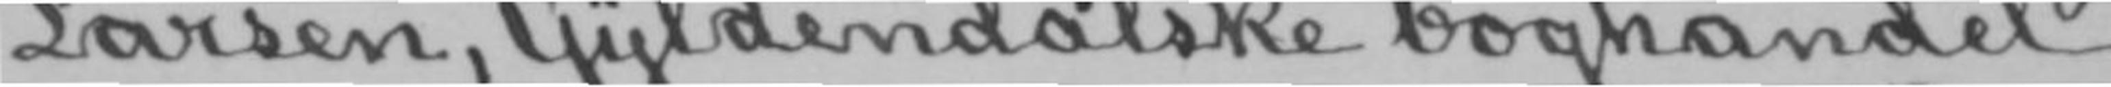Larsen, Gyldendalske boghandel,
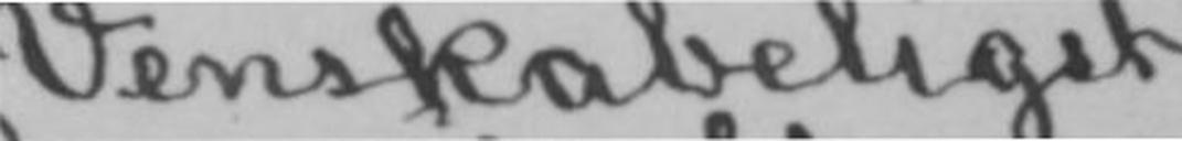Venskabeligst
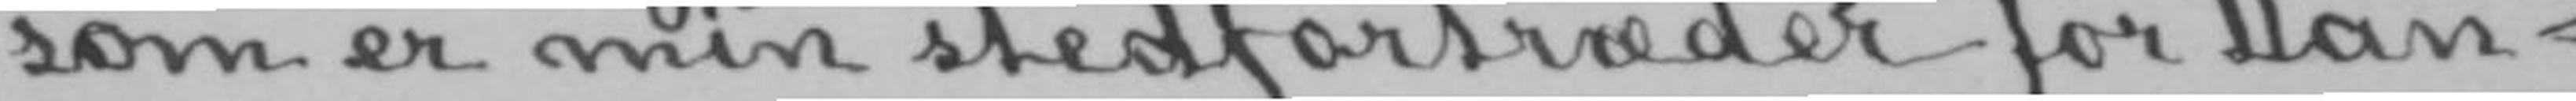som er min stedfortræder for Dan-
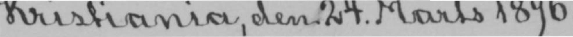Kristiania, den 24. Marts 1896.
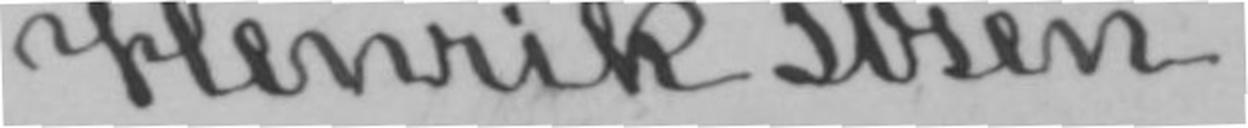Henrik Ibsen.
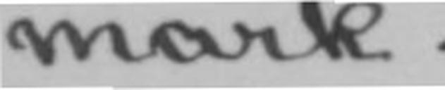mark.
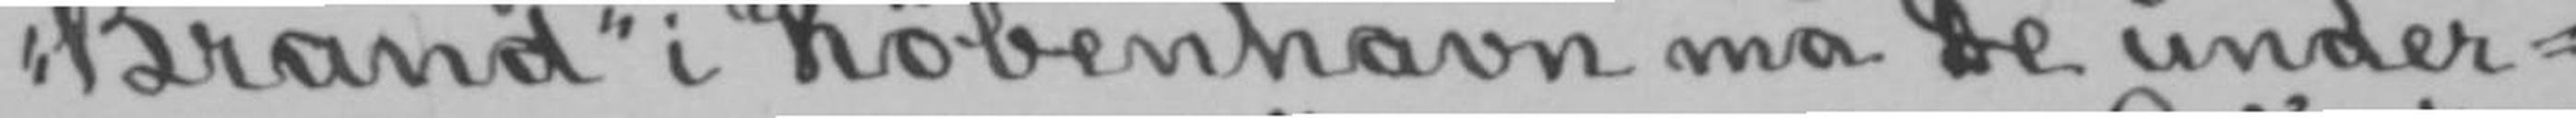«Brand» i København må De under-
